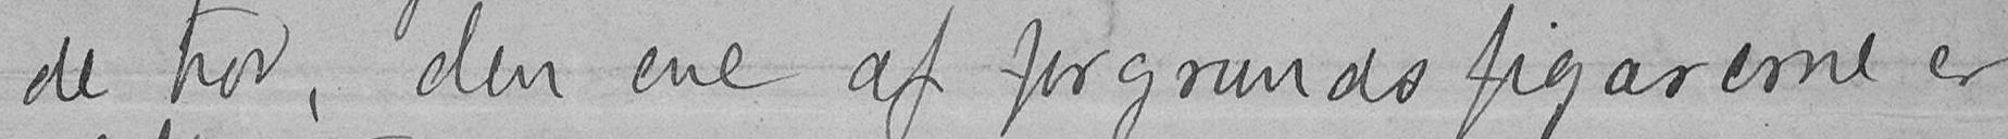de tror, den ene af forgrundsfigurerne er
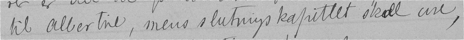til Albertine, mens slutningskapitlet skal vise,
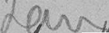dem
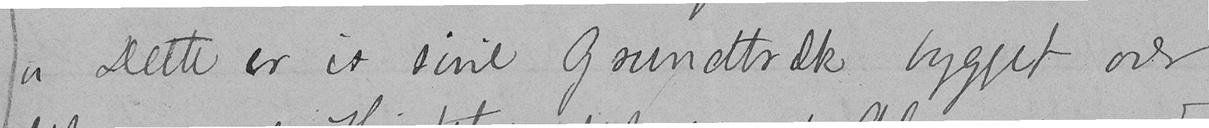"Dette er is sine Grundtræk bygget over
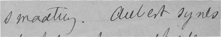smaating. Aubert synes
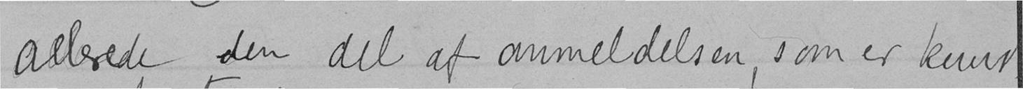Allerede den del af anmeldelsen, som er kunst-
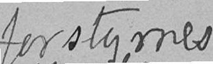forstyrres
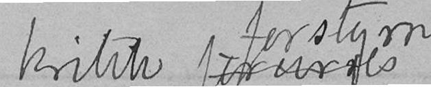kritik forvirres
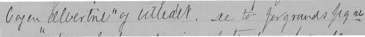bogen "Albertine" og billedet. De to forgrundsfigu-
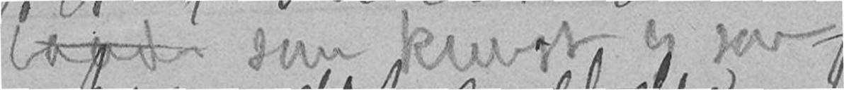baade som kunst og som
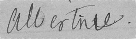Albertine.
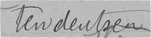tendentsen
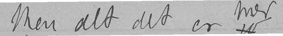Men alt det er jo
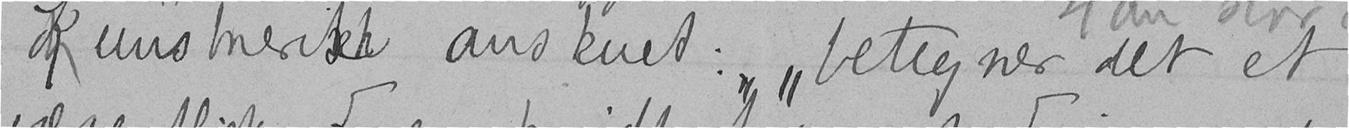Kunstnerisk anskuet: "betegner det et
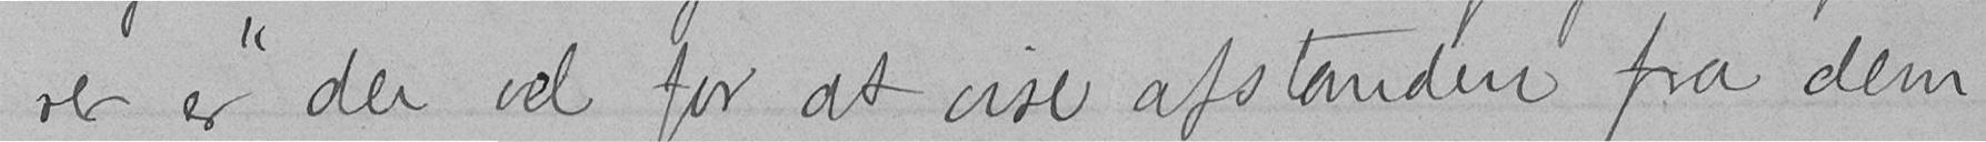rer er der vel for at vise afstanden fra dem
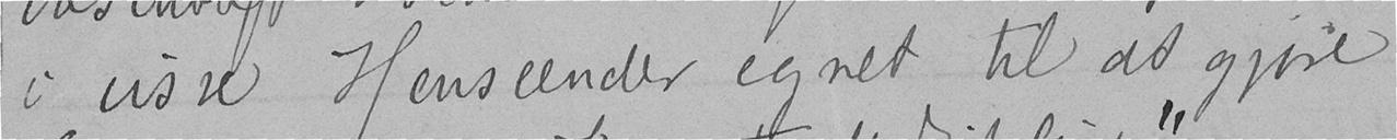i visse Henseender egnet til at gjøre
